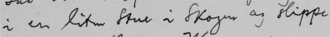i en liten Stue i Skogen og slippe
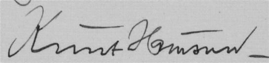Knut Hamsun.
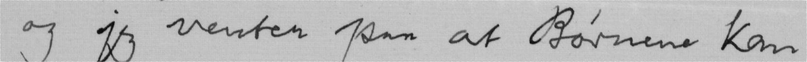og jeg venter paa at Børnene kan
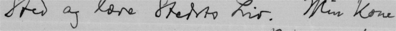Sted og lære Stedets Liv. Min Kone
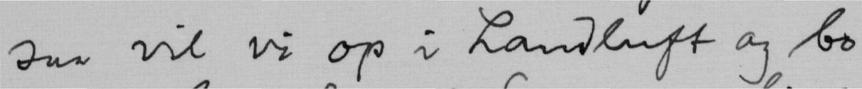saa vil vi op i Landluft og bo
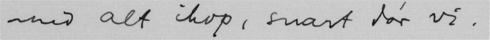med alt ihop, snart dør vi.
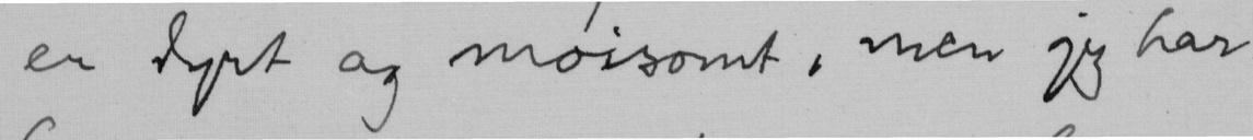er dyrt og møisomt, men jeg har
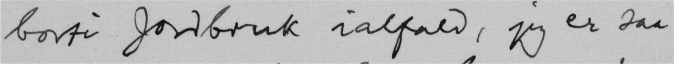borti Jordbruk ialfald, jeg er saa
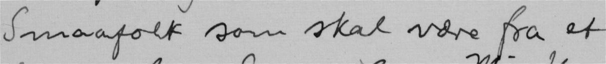Smaafolk som skal være fra et
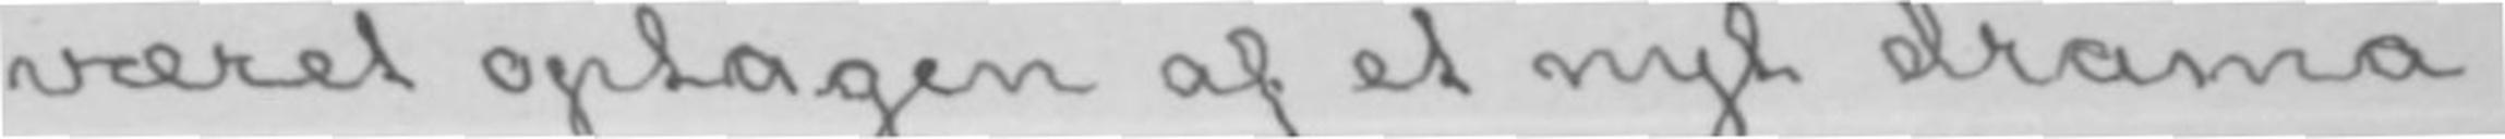været optagen af et nyt drama-
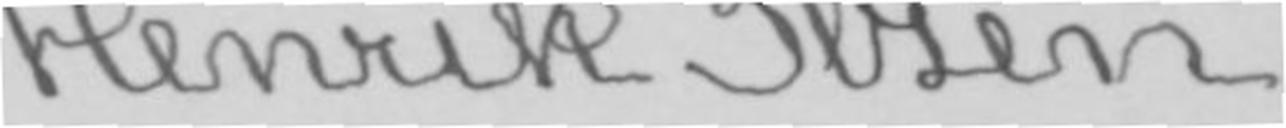Henrik Ibsen.
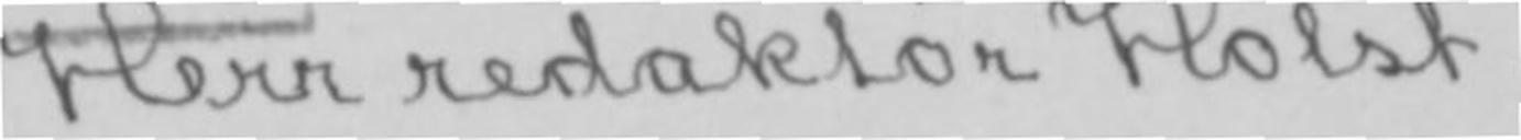Herr redaktør Holst.
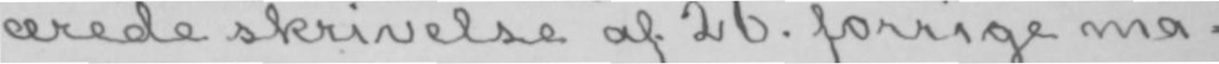ærede skrivelse af 26. forrige må-
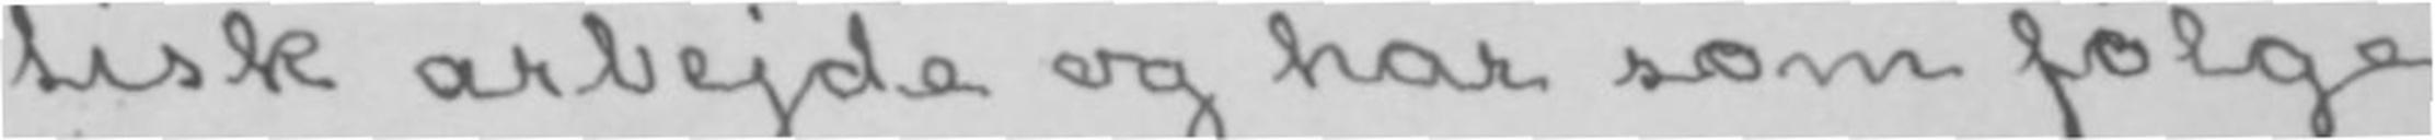tisk arbejde og har som følge
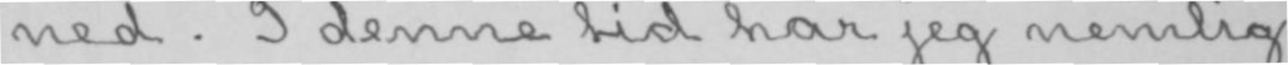ned. I denne tid har jeg nemlig
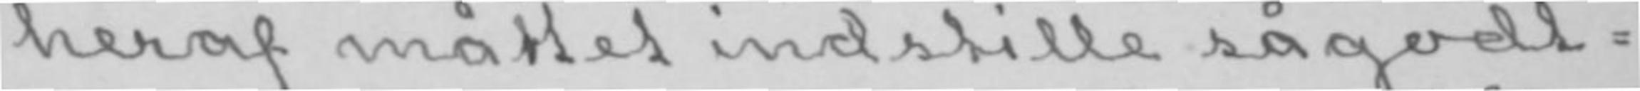heraf måttet indstille sågodt-
